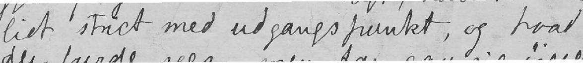lidt strict med utgangspunkt, og hvad
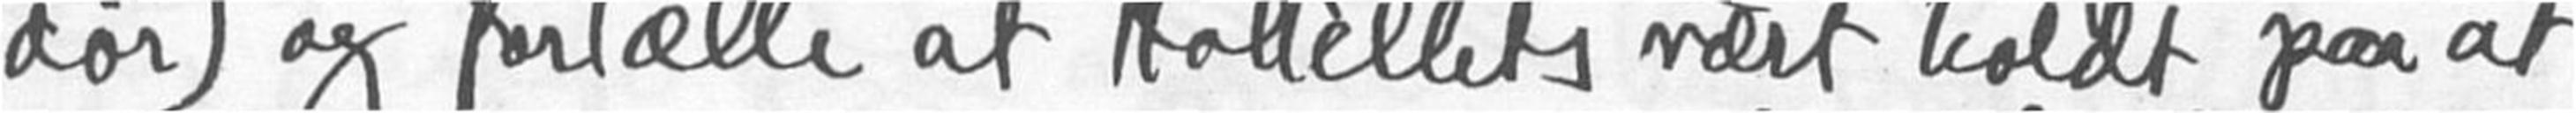dør) og fortælle at hotellets vært holdt paa at
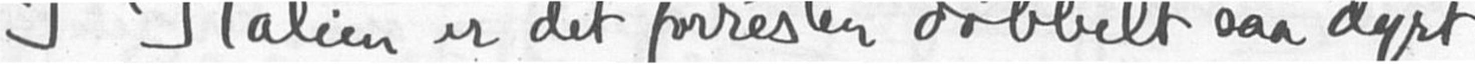I Italien er det forresten dobbelt saa dyrt
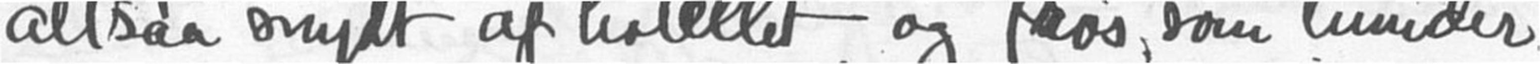altsaa snydt af hotellet - og frøs som hunder.
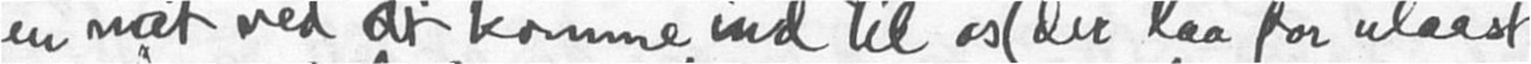en nat ved at komme ind til os (der laa for ulaast
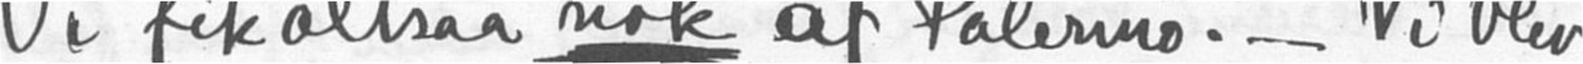Vi fik altsaa nok af Palermo. - Vi blev
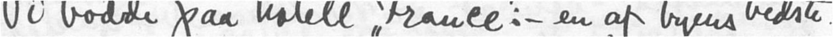Vi bodde paa hotell "France": - en af byens bedste
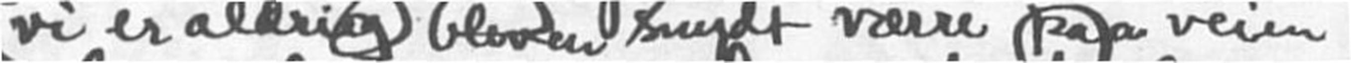(Vi er aldrig bleven snydt værre paa veien).
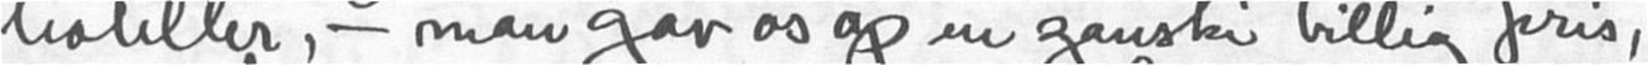hoteller, - man gav os op en ganske billig pris
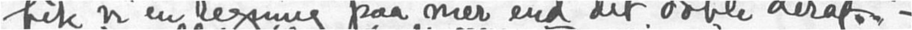fik vi en regning paa mer end det doble deraf. -
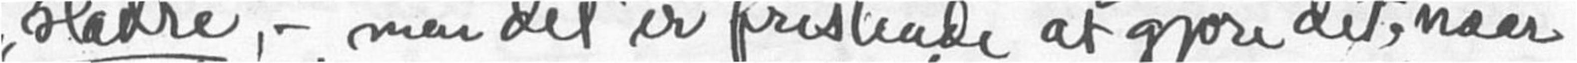"sladre", - men det er fristende at gjorde det, naar
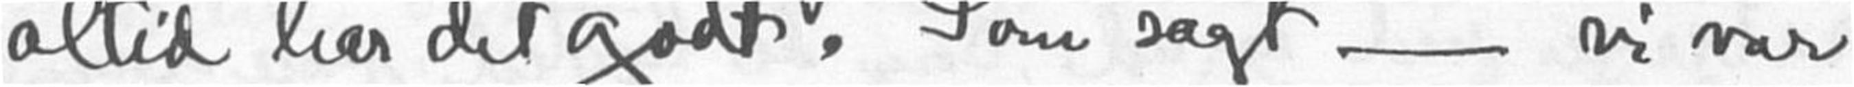altid har det godt. Som sagt - vi var
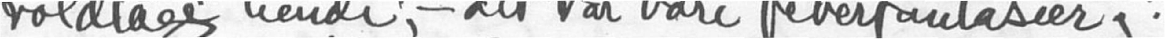voldtage hende ; - det var bare feberfantasier,
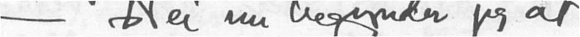Nei nu begynder jeg at
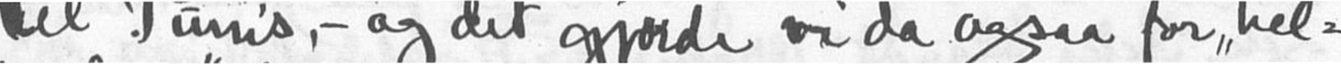til Tunis, - og det gjorde vi da ogsaa for "hel-
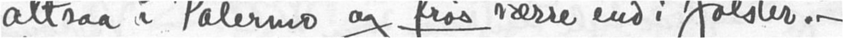altsaa i Palermo og frøs værre end i Jølster. -
